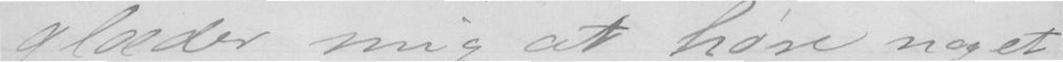glæder mig at høre noget
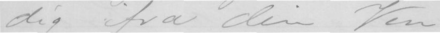dig ifra din Ven
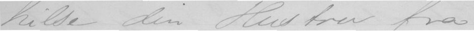hilse din Hustru fra
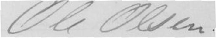Ole Olsen.
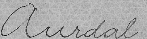Aurdal
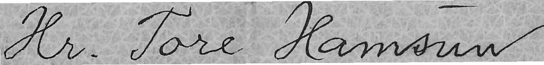Hr. Tore Hamsun
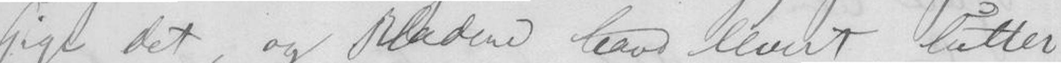sige det, og Bladene have levert lutter
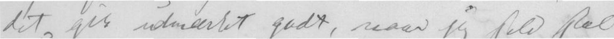det gik udmærket godt, naar jeg selv skal
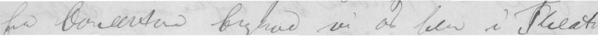fra Concerten begave vi os her i Theatret,
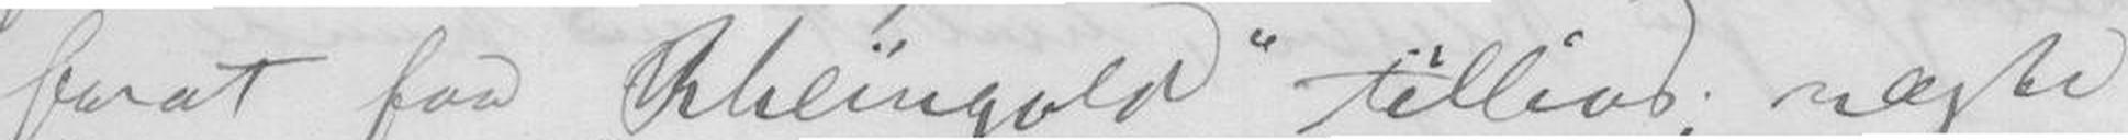forat faa Rheingold tillivs, næste
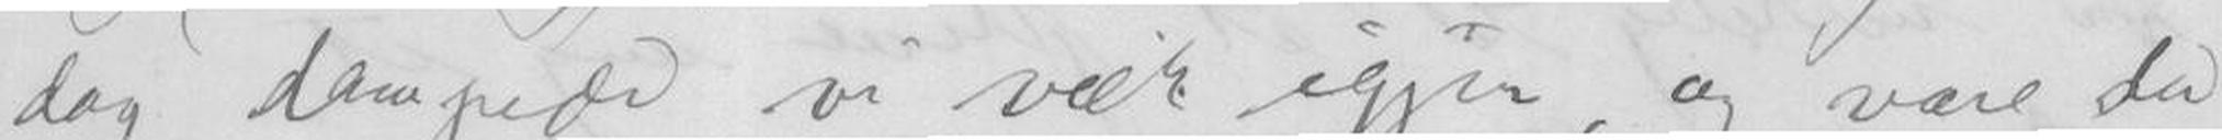dag dampede vi væk igjen, og vare da
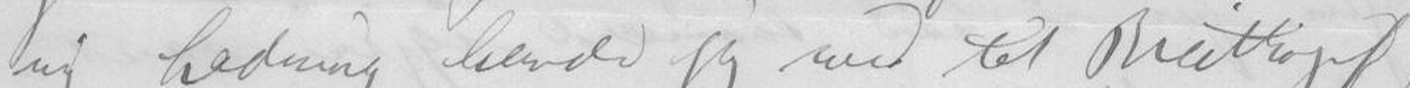ny Ladning havde jeg med til Breitkopf,
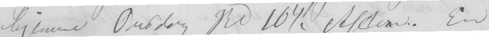hjemme Onsdag Kl 10 1/2 Aften. En
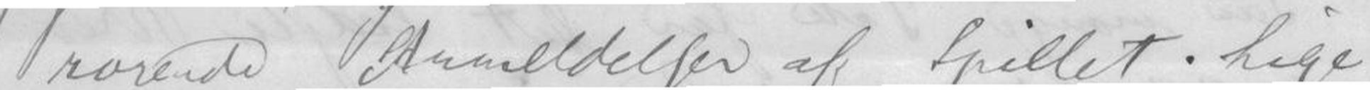rosende Anmeldelser af Spillet. Lige
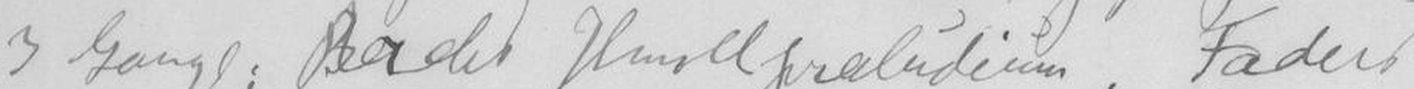3 Gange; Bachs Hmollpræludium, Faders
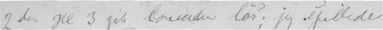2den Kl 3 gik Concerten løs; jeg spillede
